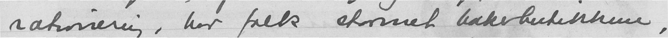rationering, har folk stormet bakerbutikkene,
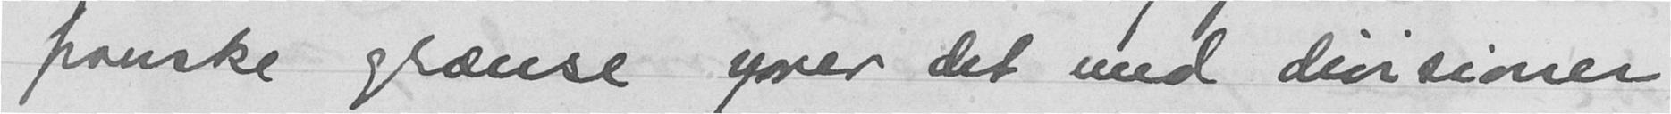franske grænse yrer det med dicisioner
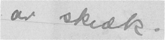av skræk.
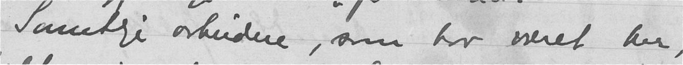Samtlige arbeidere, som har været her,
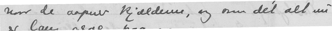naar de aapner kjælderne, og som det alt nu
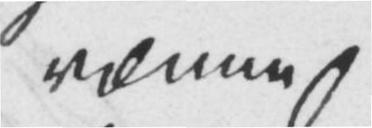vænne
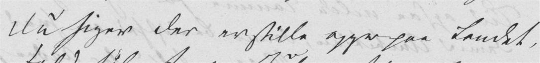Du siger der er stille oppe paa Landet,
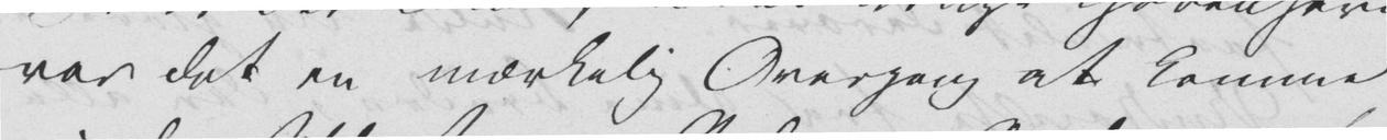var det en mærkelig Overgang at komme
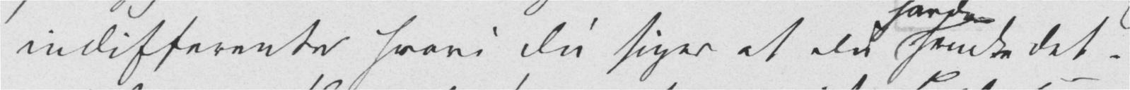indifferente hvori Du siger at Du sendte det.
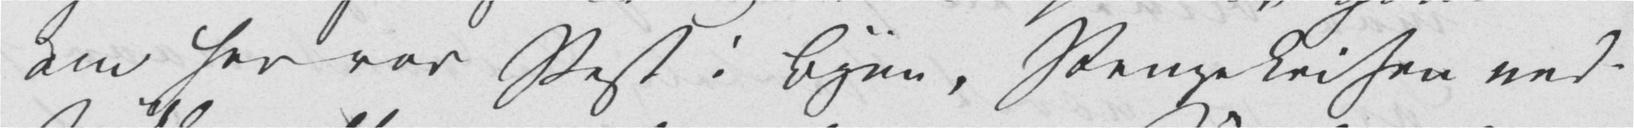om her var Pest i Byen, Pengekrisen ned-
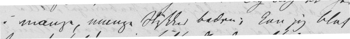i mange, mange Stykker bedre; kan jeg blot
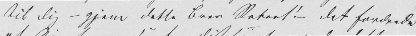til Dig – gjem dette Brev Robert! – det fordrede
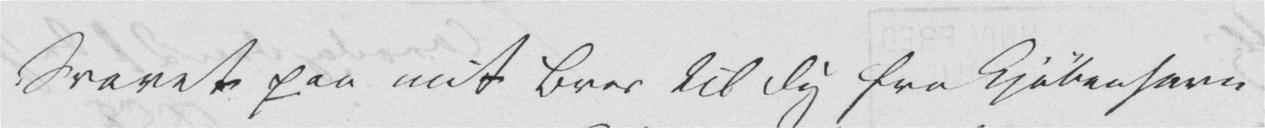Svaret paa mit Brev til Dig fra Kjøbenhavn
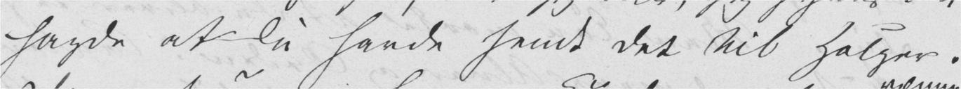sagde at Du havde sendt det til Holger.
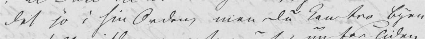det jo i sin Orden, men Du kan tro Byen
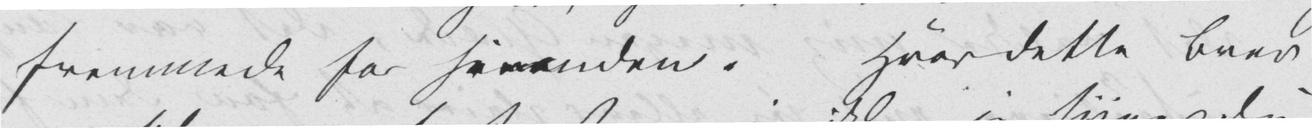fremmede for hinanden. Hvor dette Brev
